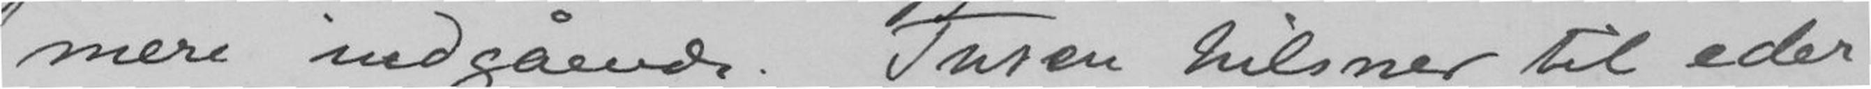mere indgående. Tusen hilsner til eder
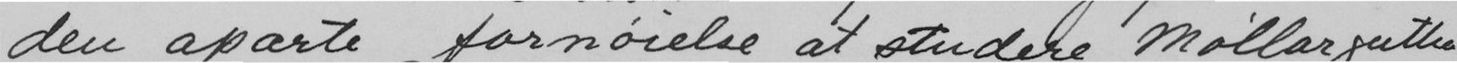den aparte fornøielse at studere Møllargutten
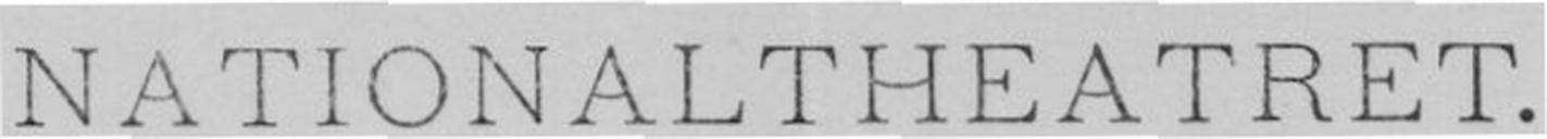NATIONALTHEATRET.
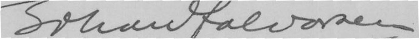Johan Halvorsen
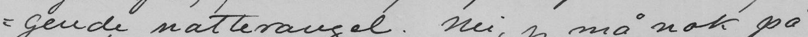gende natterangel. Nei, jeg må nok på
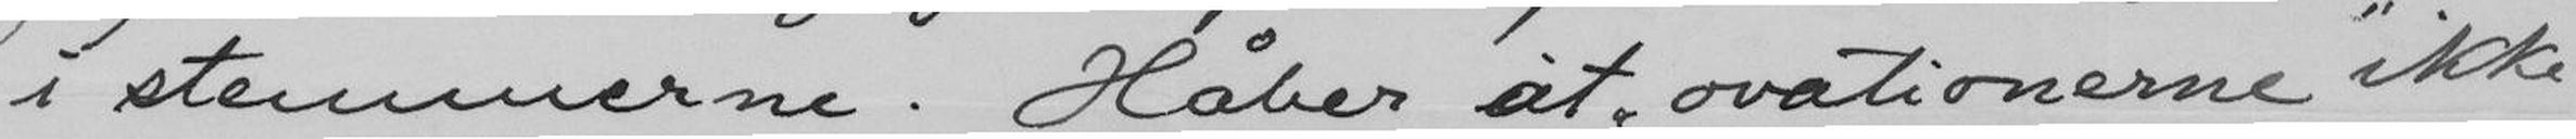i stemmerne. Håber at ovationerne ikke
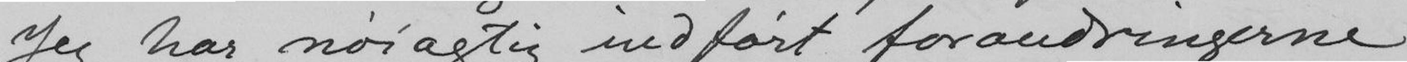Jeg har nøiagtig indført forandringerne
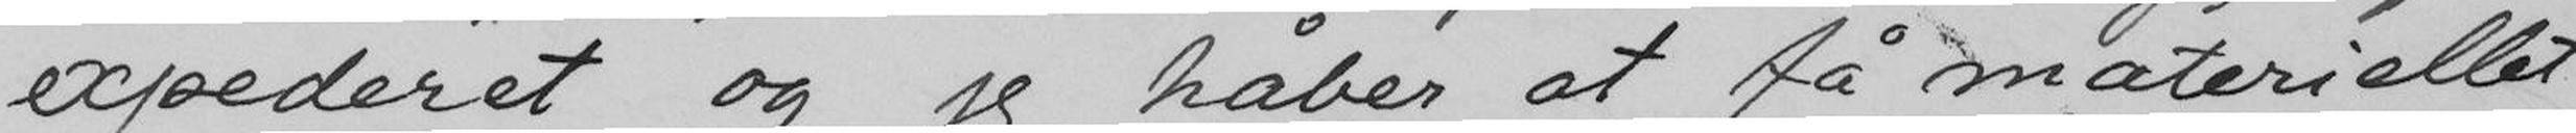expederet og jeg håber at få materiellet
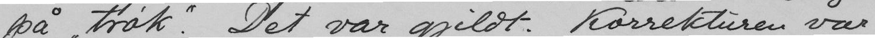på trøk. Det var gjildt. Korrekturen var
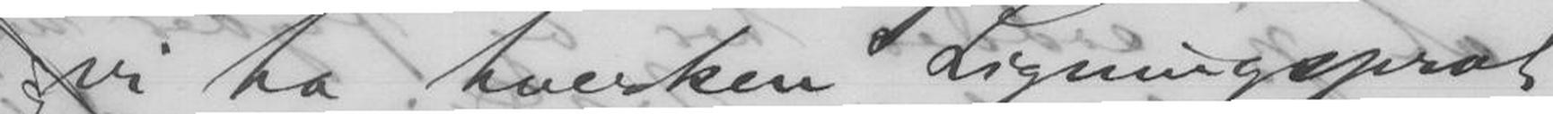vi ha hverken Ligningsprat
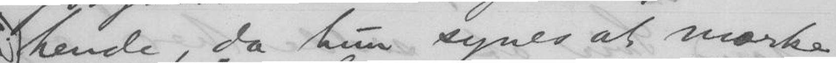hende, da hun synes at mærke,
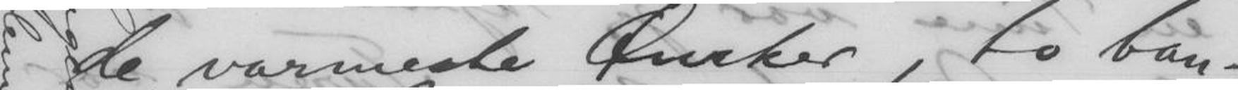de varmeste Ønsker, to ban-
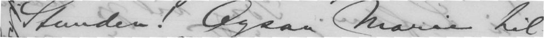Stunden! Ogsaa Marie hil-
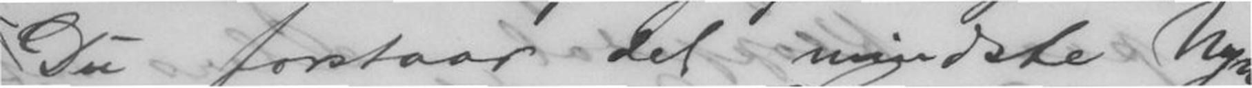Du forstaar det mindste Nyn
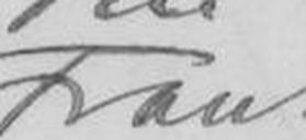Frants.
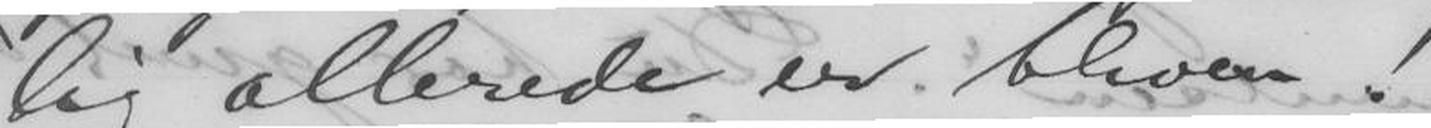lig allerede er bleven!
